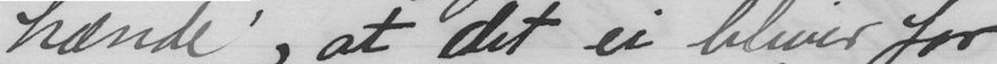hænde', at det ei bliver for
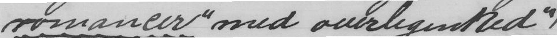romancer "med overlegenthed""
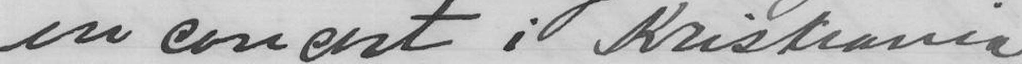en concert i Kristiania
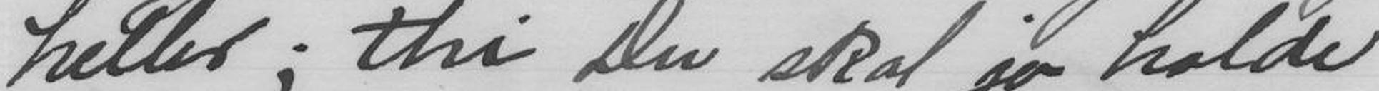heller; thi Du skal jo holde
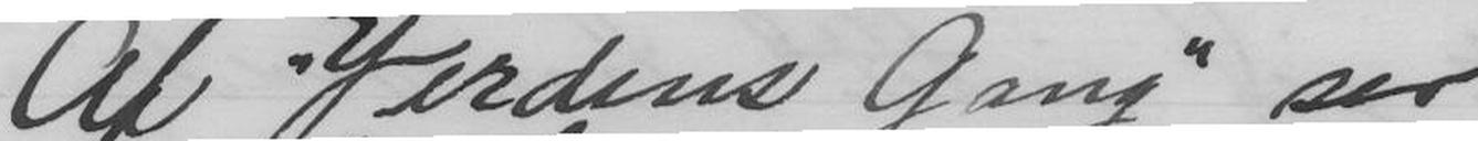Af "Verdens Gang" ser
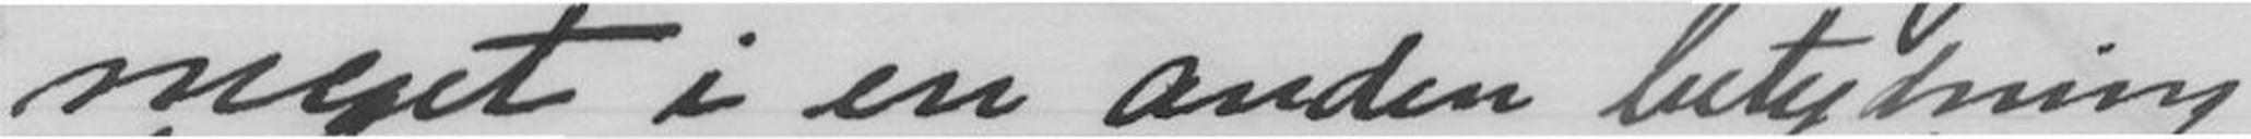meget i en anden betydning
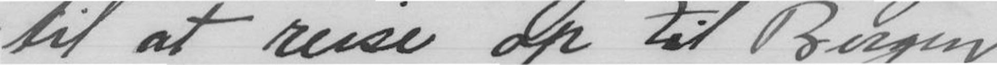til at reise op til Bergen
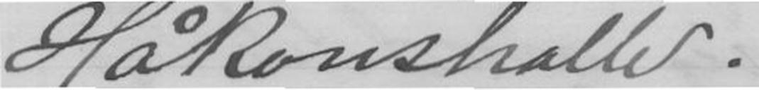Håkonshalld.
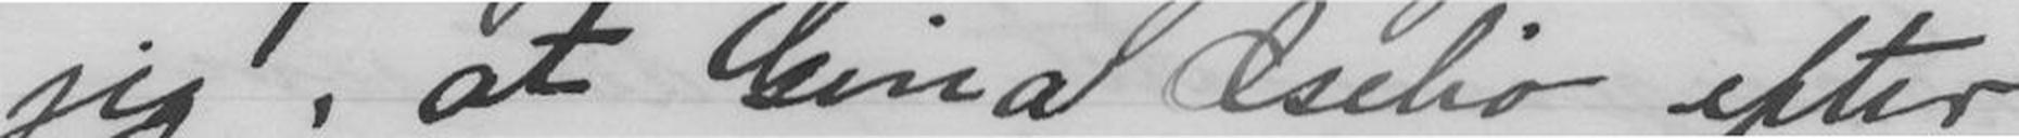jeg, at Gina Oselio efter
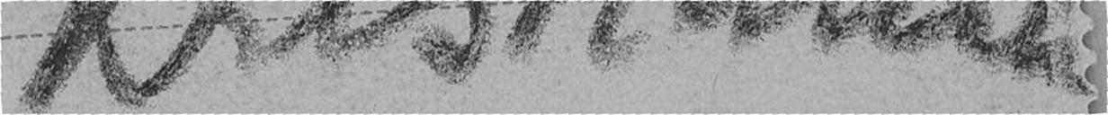Kristiania
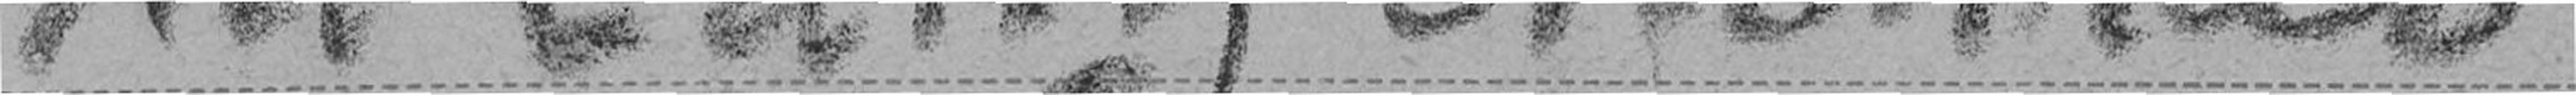Fru Cally Monrad
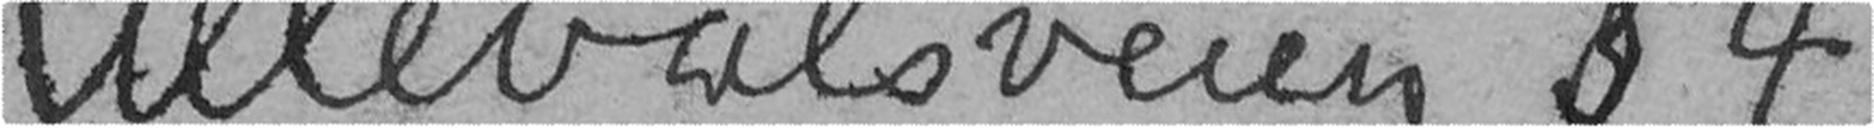Ullevålsveien 54
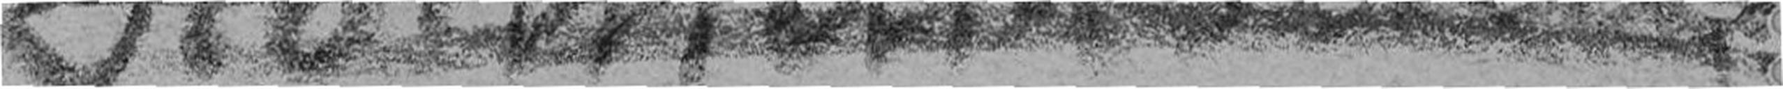Holmenkollen
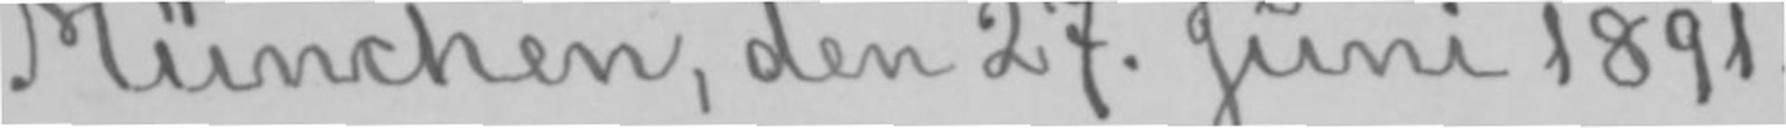München, den 27. Juni 1891.
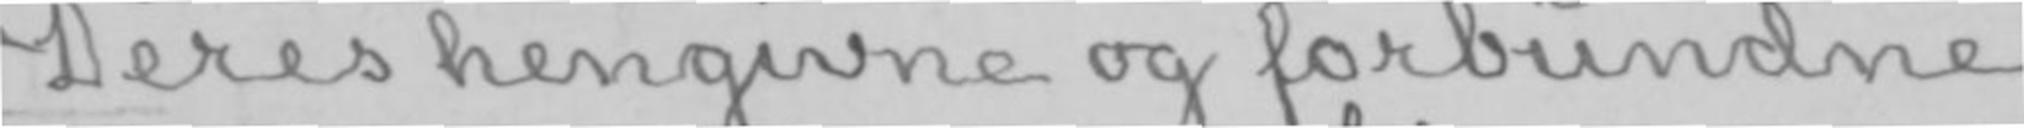Deres hengivne og forbundne
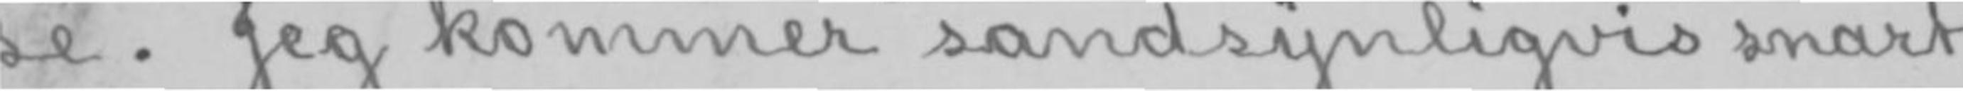se. Jeg kommer sandsynligvis snart
